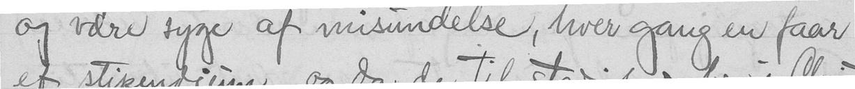og være syge af misundelse, hver gang en faar
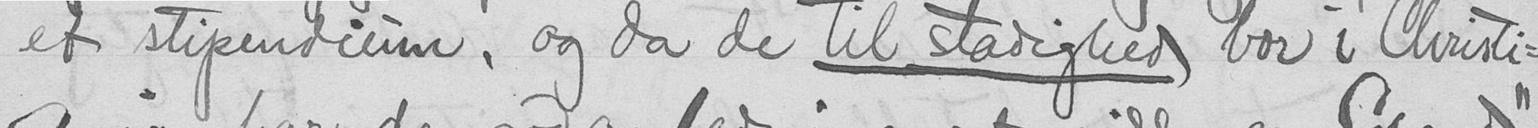et stipendium, og da de tilstadighed bor i Christi-
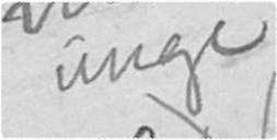unge
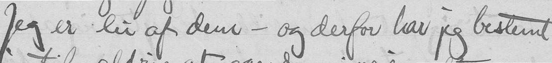Jeg er lei af dem - og derfor har jeg bestemt
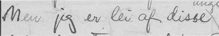Men jeg er lei af disse
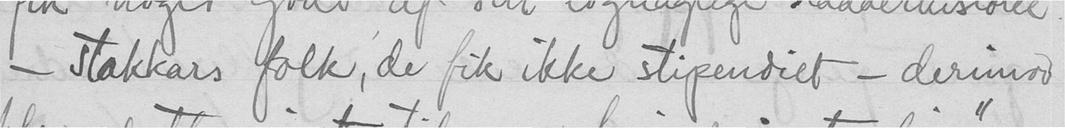- stakkars folk, de fik ikke stipendiet - derimod
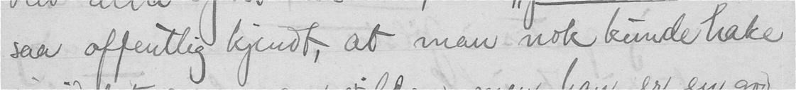saa offentlig kjendt, at man nok kunde hake
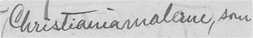Christianiamalerne, som
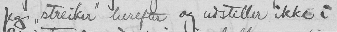Jeg "streiker" herefter og udstiller ikke i
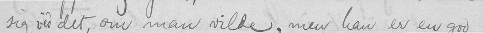sig ved det, om man vilde, men han er en god
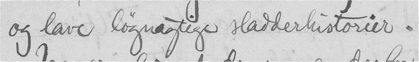og lave løgnagtige sladderhistorier.
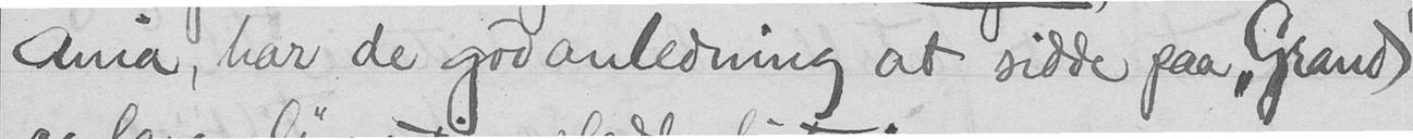ania, har de god anledning at sidde paa "Grand"
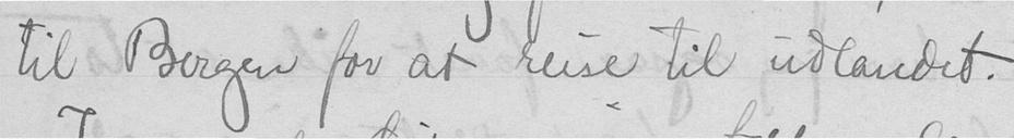til Bergen - for at reise til udlandet.
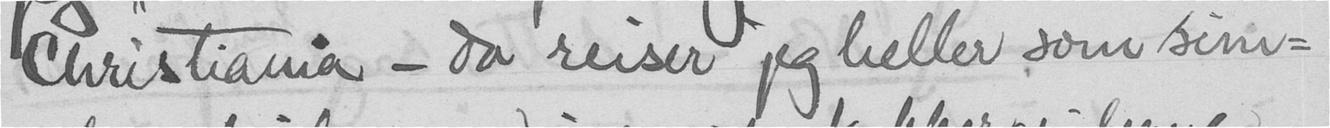Christiania - da reiser jeg heller som sim-
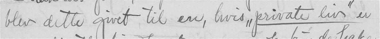blev dette givet til en, hvis "private liv" er
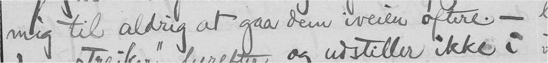mtig til aldrig at gaa dem iveien oftere. -
